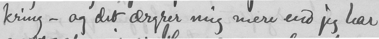kring - og det ærgrer mig mere end jeg har
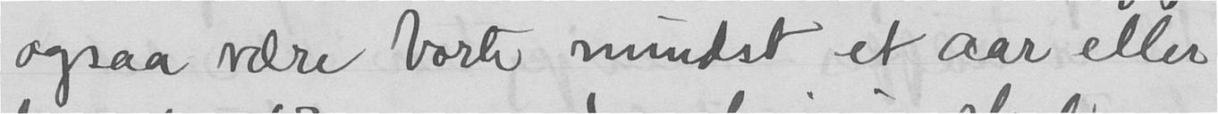ogsaa være borte mindst et aar eller
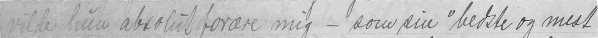vilde hun absolut forære mig - som sin "bedste og mest
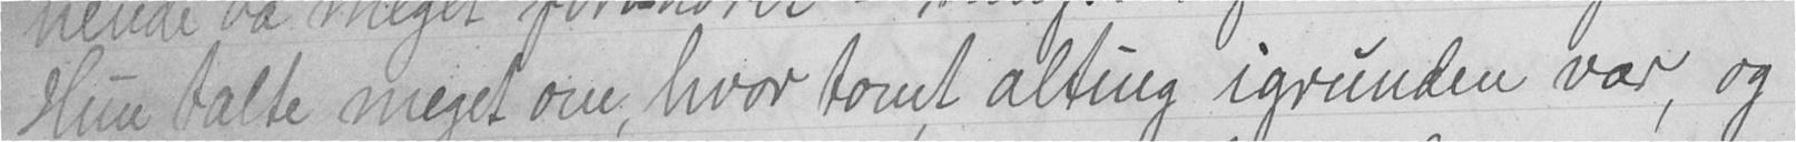Hun talte meget om, hvor tomt alting igrunden var, og
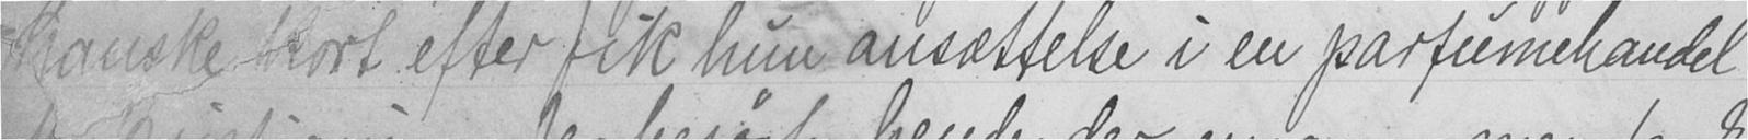Ganske kort efter fik hun ansættelse i en parfumehandel
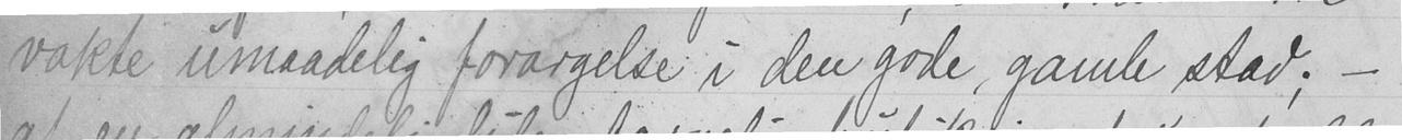vakte umaadelig forargelse i den gode, gamle stad; -
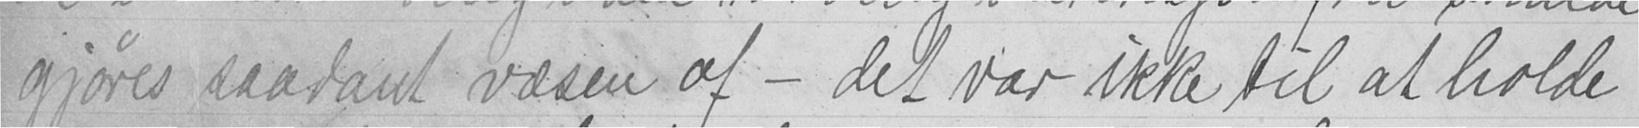gjøres saadant væsen af - det var ikke til at holde
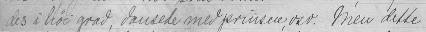des i høi grad, dansede med prinsen, osv. Men dette
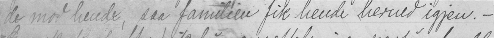de mod hende, saa familien fik hende herned igjen. -
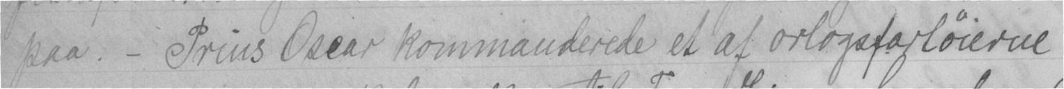paa. - Prins Oscar kommanderede et af orlogsfartøierne
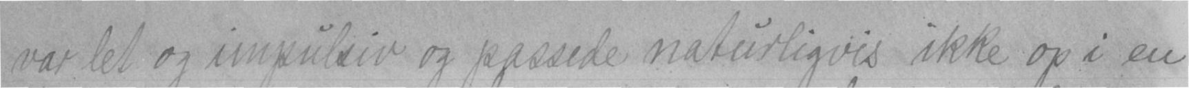var let og impulsiv og passede naturligvis ikke op i en
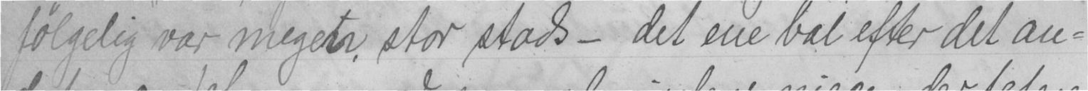følgelig var meget stor stads - det ene bal efter det an-
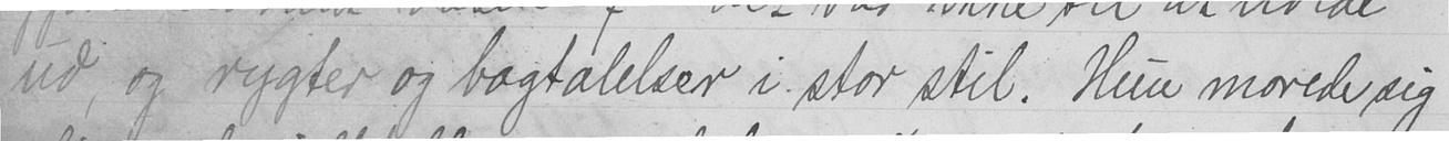ud, og rygter og bagtalelser i stor stil. Hun morede sig
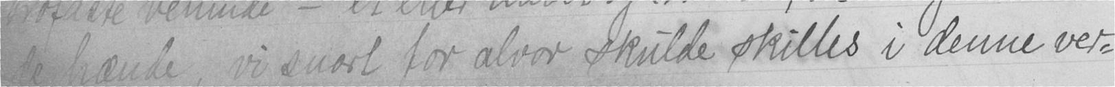de hænde; vi snart for alvor skulde skilles i denne ver-
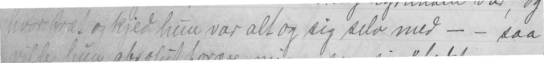hvor træt og kjed hun var alt og sig selv med - - saa
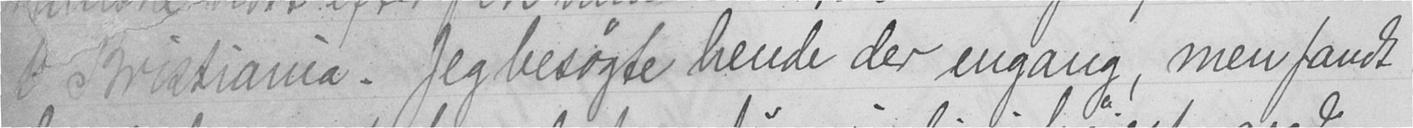i Kristiana. Jeg besøgte hende der engang, men fandt
